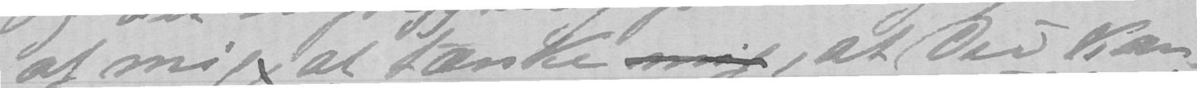af mig, at tænke mig, at Du kan-
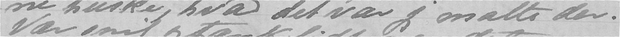ne huske, hvad det var jeg malte der.
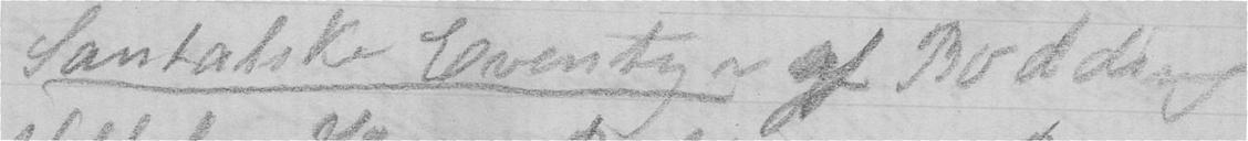Santalske Eventyr af Bodding
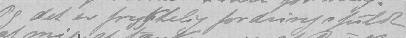Og det er fryktelig fordringsfuldt
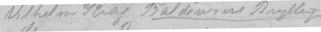Vilhelm Krag Baldevins Bryllup
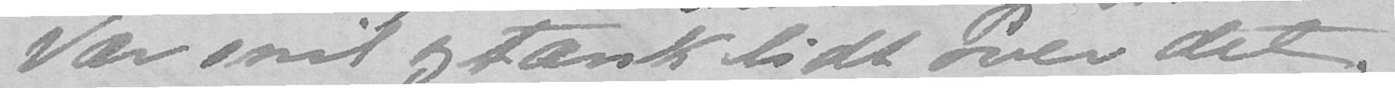Vær snil og tænk lidt over det.
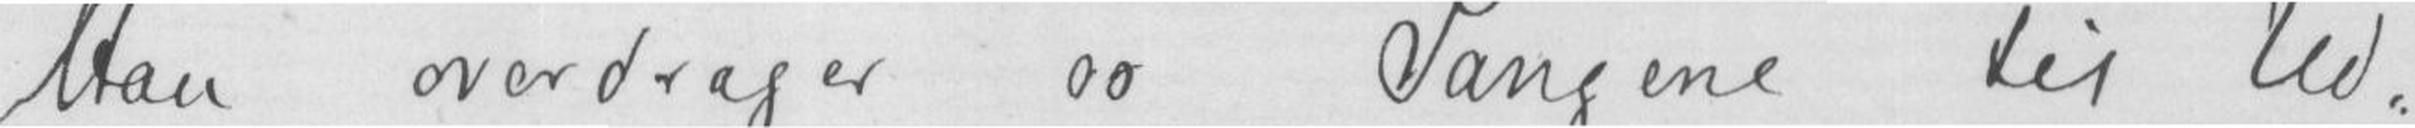Han overdrager os Sangene til Ud-
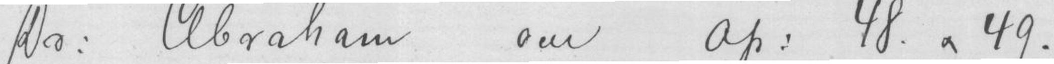Dr. Abraham om op: 48 a 49.
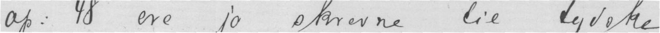op: 48 ere jo skrevet til tydoke
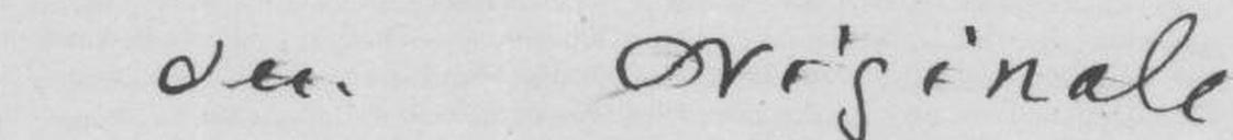den orginale
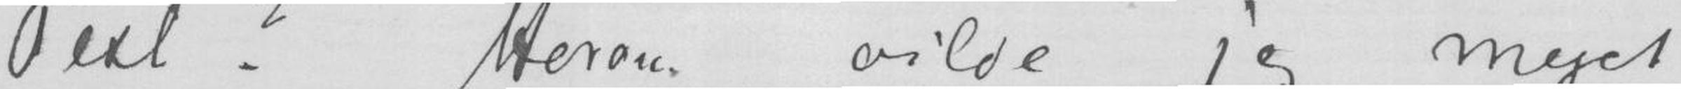Text? Herom vilde jeg meget
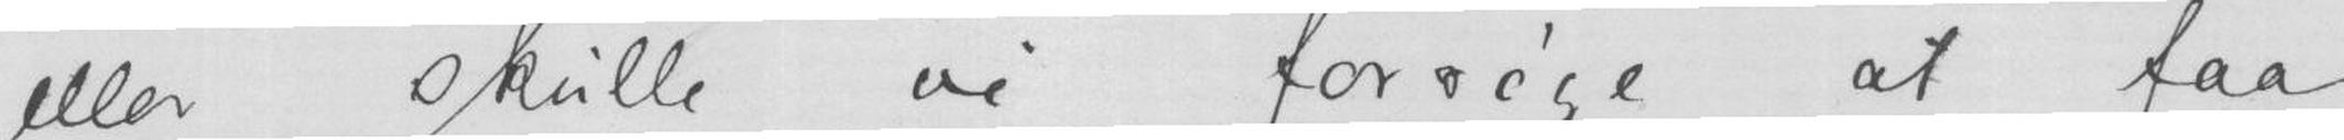eller skulle vi forsøge at faa
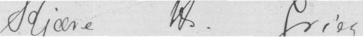Kjære Hr. Grieg
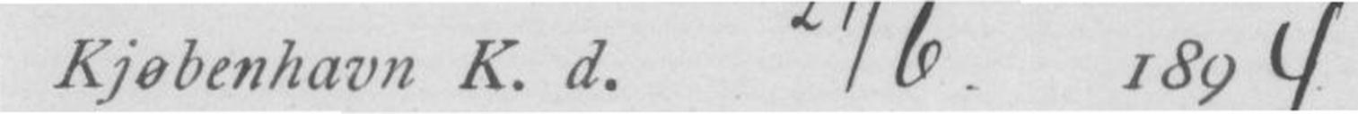Kjøbenhavn K.d. 27/6 1894
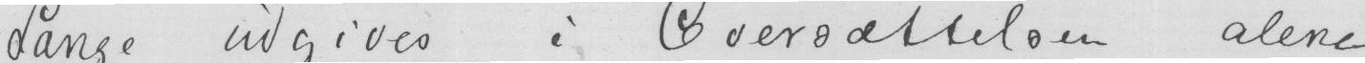Sange udgives i Oversættelsen alene
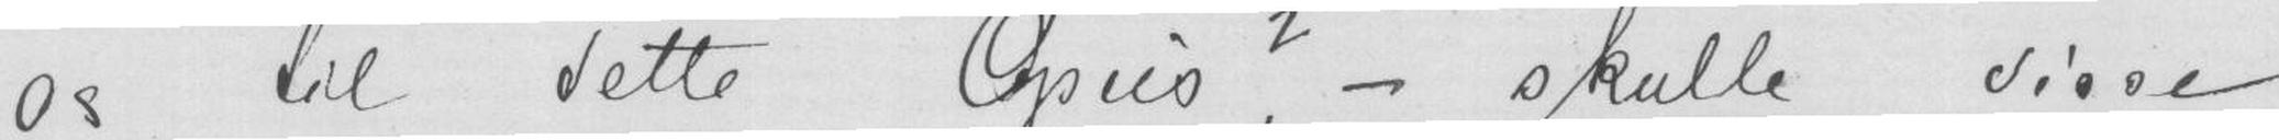os til dette Opus? - skulle disse
